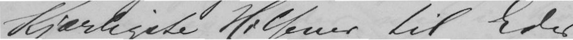Kjærligste Hilsener til Eder
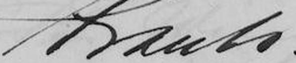Frants.
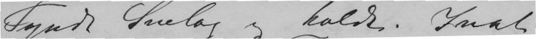Tyndt Snelag og koldt. Inat
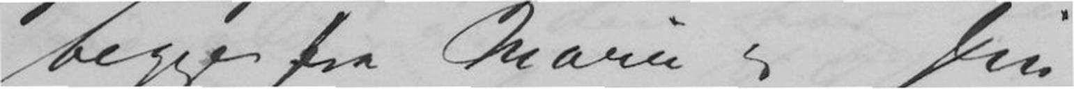begge fra Marie og Din
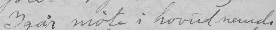Igår möte i hovud-nemda
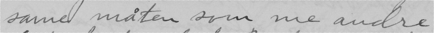same måten som me andre
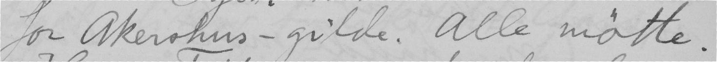for Akershus-gilde. Alle mötte.
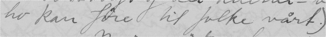ho kan före til folke vårt.
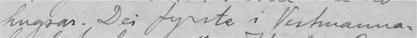hugsar. Dei fyrste i Vestmanna-
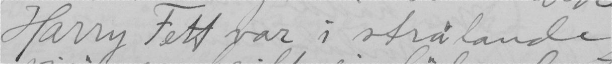Harry Fett var i strålande
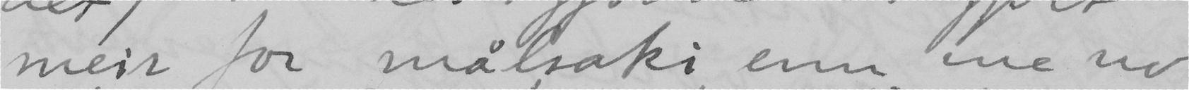meir for målsaki enn me no
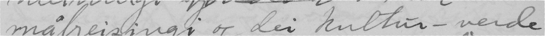målreisingi og dei kultur-verde
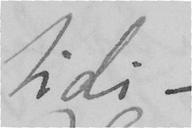tidi –
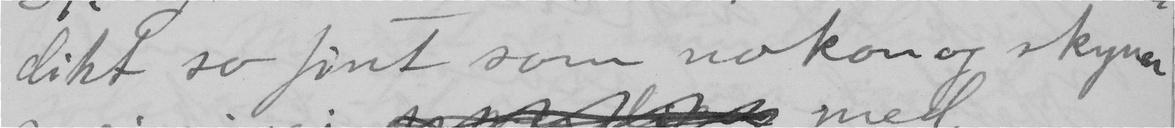dikt so fint som nokon og skyner
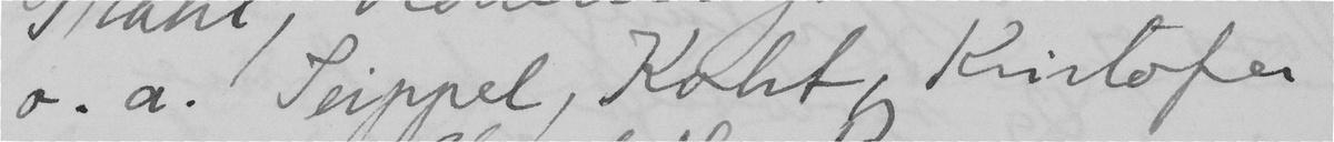o.a. Seippel, Koht, Kristofer
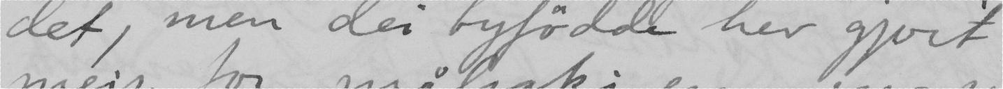det, men dei byfödde hev gjort
